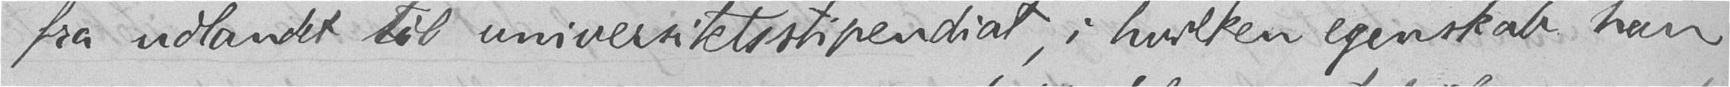fra udlandet til universitetsstipendiat, i hvilken egenskab han
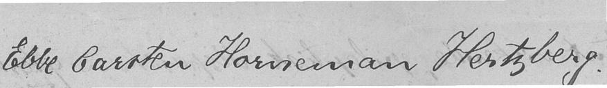Ebbe Carsten Horneman Hertzberg.
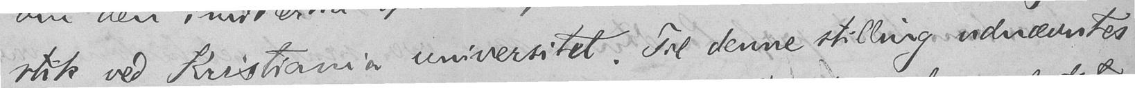stik ved Kristiania universitet. Til denne stilling udnævntes
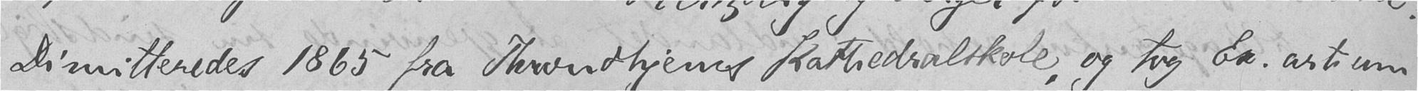Dimitteredes 1865 fra Throndhjems Kathedralskole, og tog Ex. artium
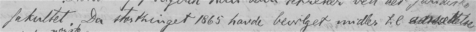fakultet. Da storthinget 1865 havde bevilget midler til ansættelse
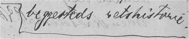beggesteds retshistorie.
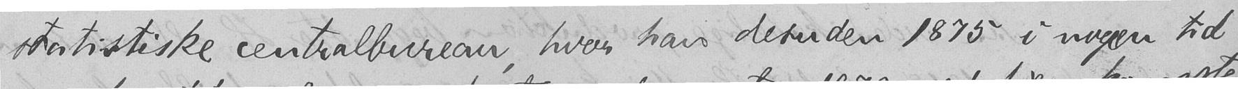statistiske centralbureau, hvor han desuden 1875 i nogen tid
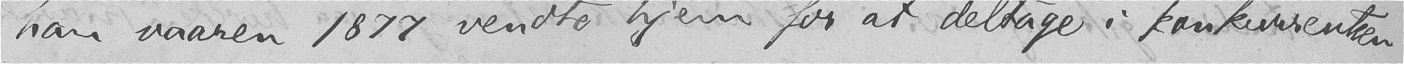han vaaren 1877 vendte hjem for at deltage i konkurrentsen
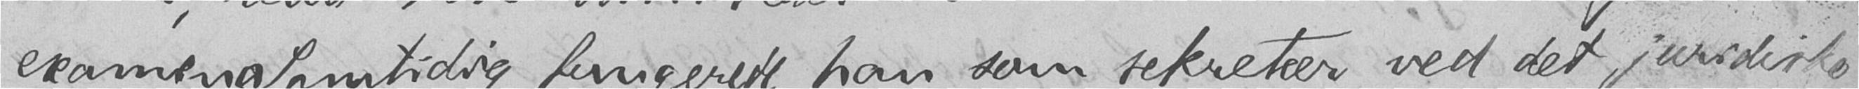examen. Samtidig fungerede han som sekretær ved det juridiske
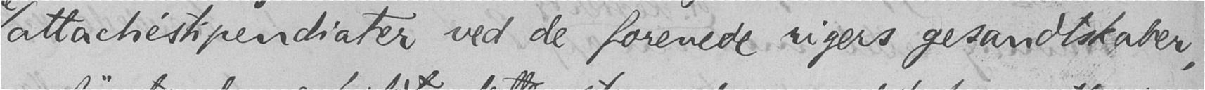attachéstipendiater ved de forenede rigers gesandtskaber,
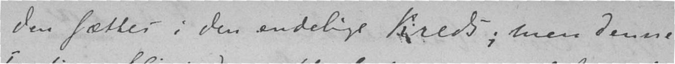den sættes i den endelige Kreds; men denne
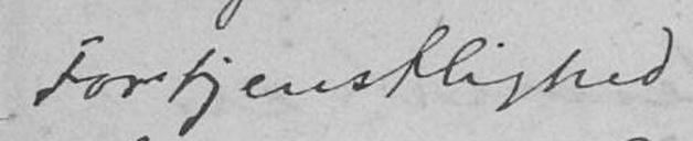Fortjenstlighed
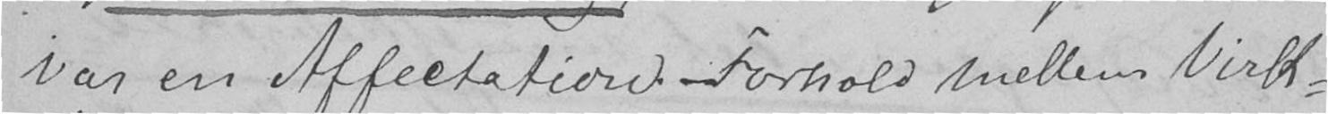var en Affectation. Forhold mellem Virk-
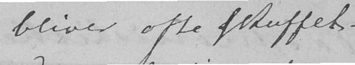bliver ofte skuffet –
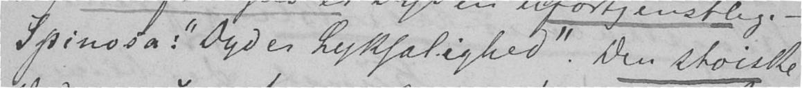Spinoza: "Dyd er Lyksalighed". Den stoiske
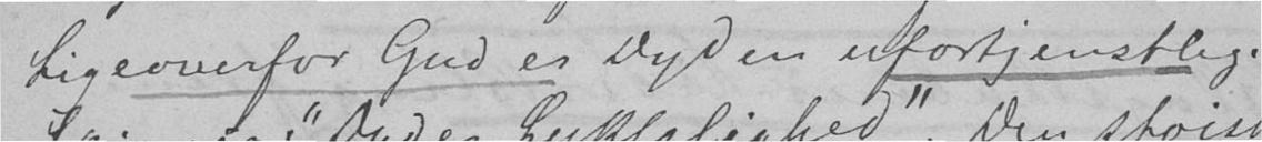Ligeoverfor Gud er Dyden ufortjenstlig.
